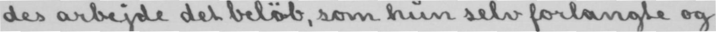des arbejde det beløb, som hun selv forlangte og
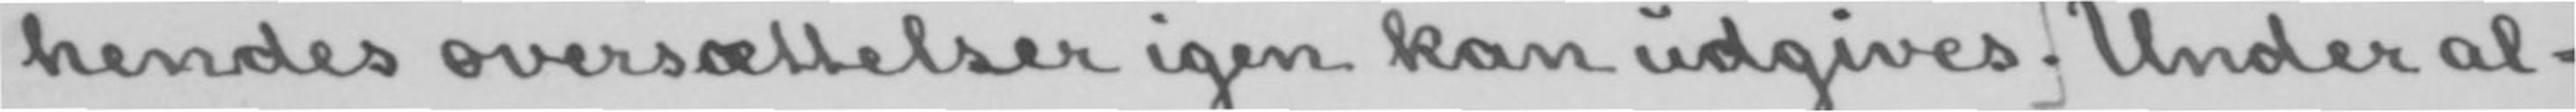hendes oversættelser igen kan udgives. Under al-
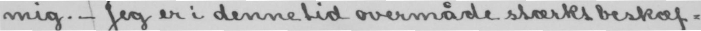mig.- Jeg er i denne tid overmåde stærkt beskæf-
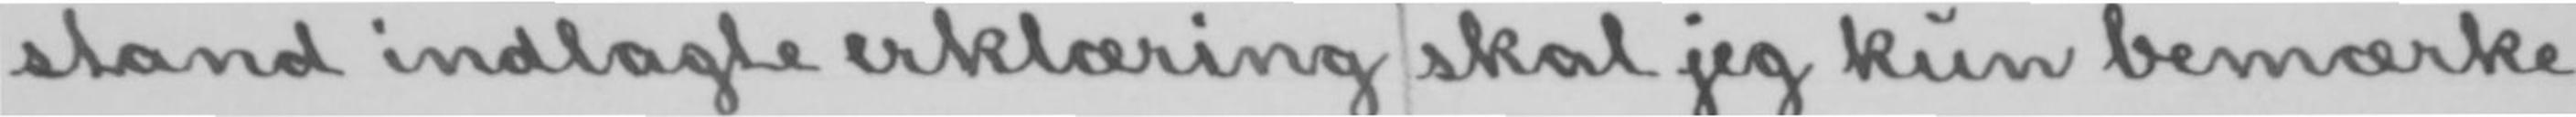stand indlagte erklæring skal jeg kun bemærke
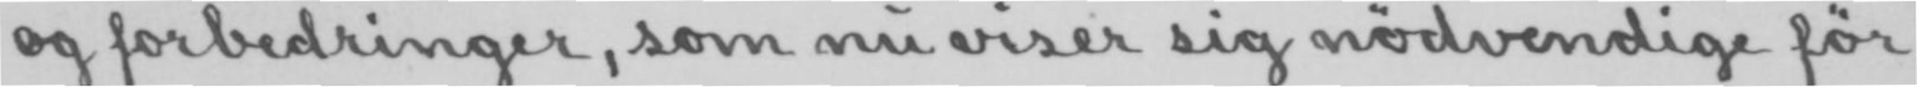og forbedringer, som nu viser sig nødvendige før
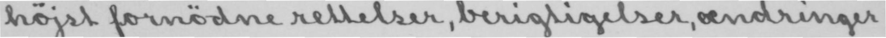højst fornødne rettelser, berigtigelser, ændringer
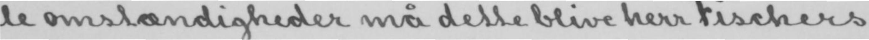le omstændigheder må dette blive herr Fischers
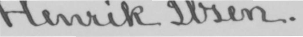Henrik Ibsen.
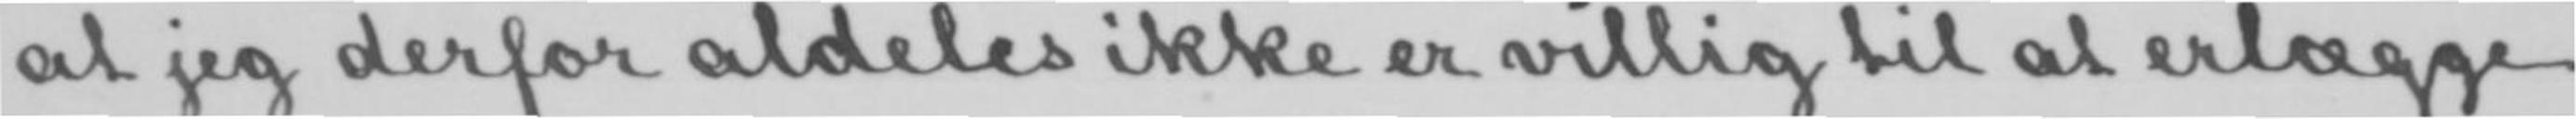at jeg derfor aldeles ikke er villig til at erlægge
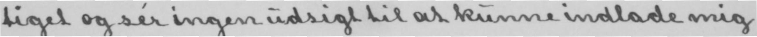tiget og sér ingen udsigt til at kunne indlade mig
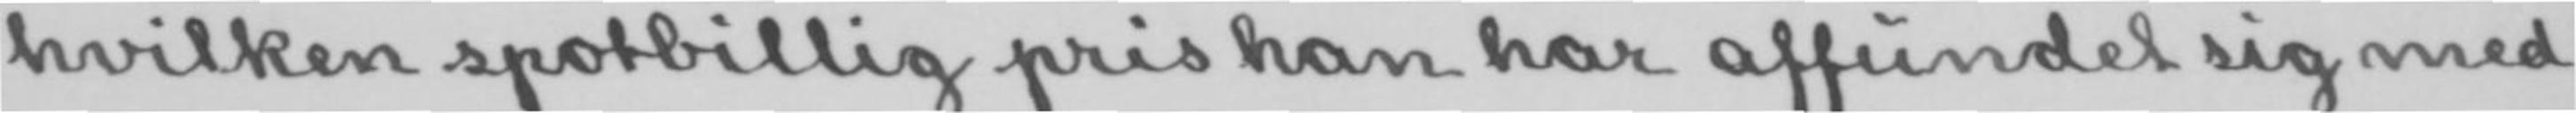hvilken spotbillig pris han har affundet sig med
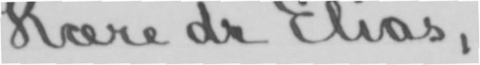Kære dr Elias,
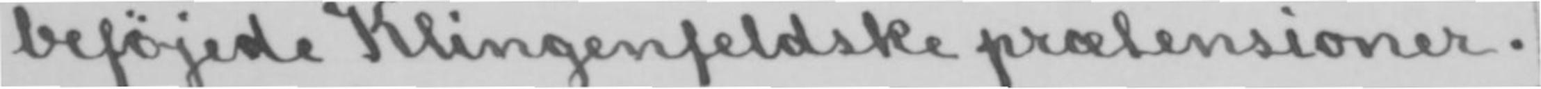beføjede Klingenfeldske prætensioner.
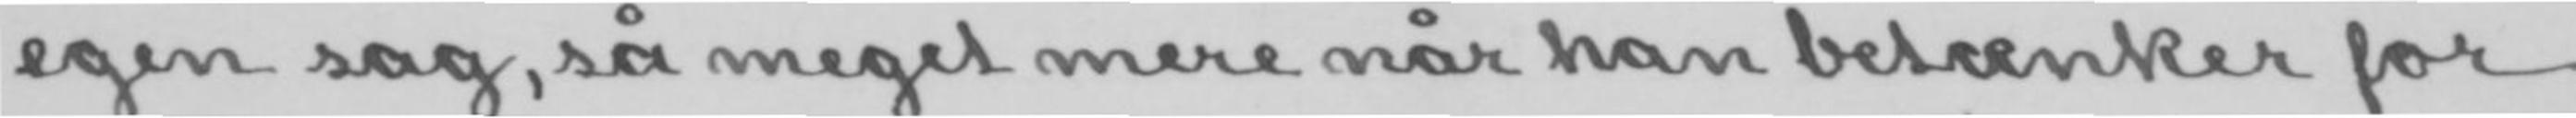egen sag, så meget mere når han betænker for
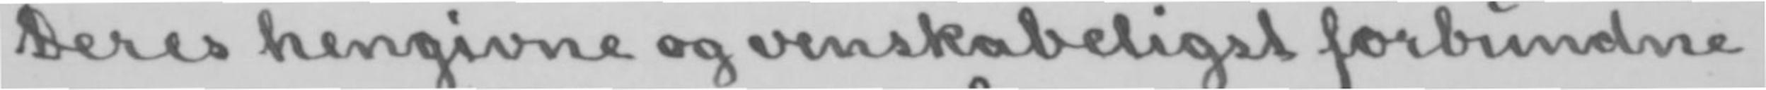Deres hengivne og venskabeligst forbundne
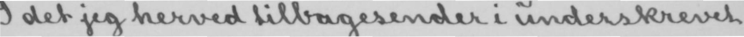I det jeg herved tilbagesender i underskrevet
